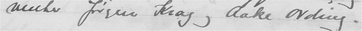venter Jørgen Krag og Aake Ording.
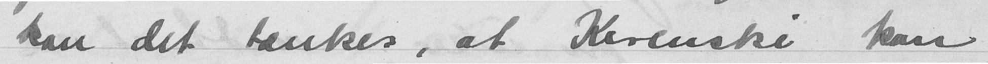kan det tænkes, at Kerenski kan
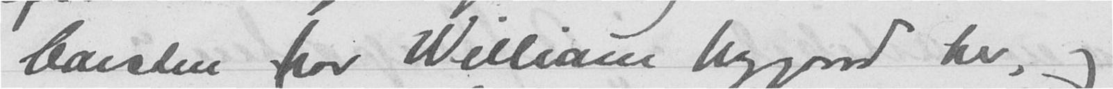Carsten for William Nygaard her, og
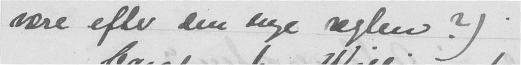være efter den nye reglen?).
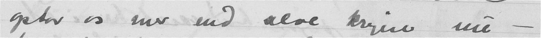optar os mer end selve krigen nu -
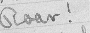Roar!
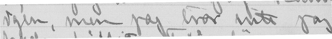igen, men jag tror inte jag
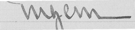Ingem
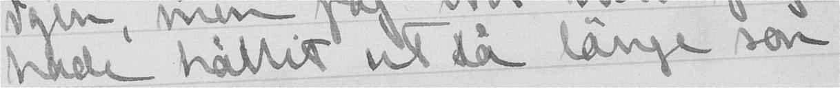hade hållit ut så länge som
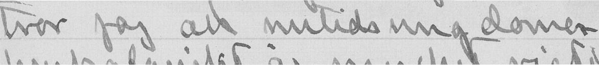tror jag alt nutids ungdomer
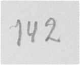142
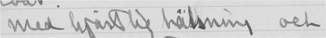med hjartlig hälsning det
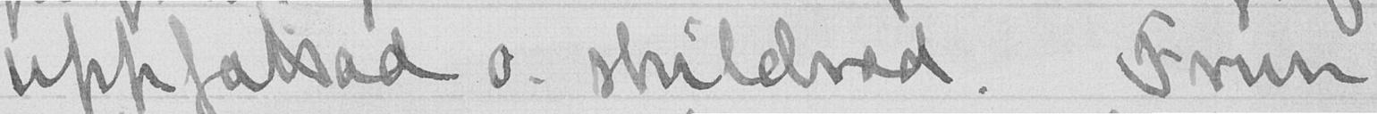uppjatsaa o. skildrad. Frun
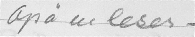Også en leser -
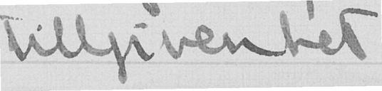tillgivenhet
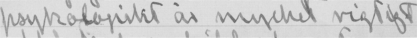psykologiskhet är mychet vigtigt
